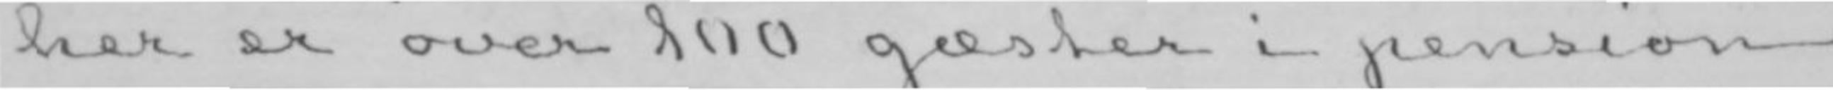her er over 100 gæster i pension
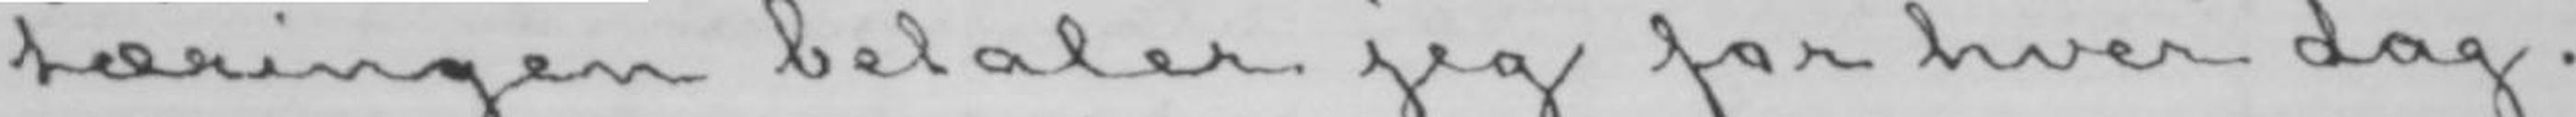tæringen betaler jeg for hver dag.
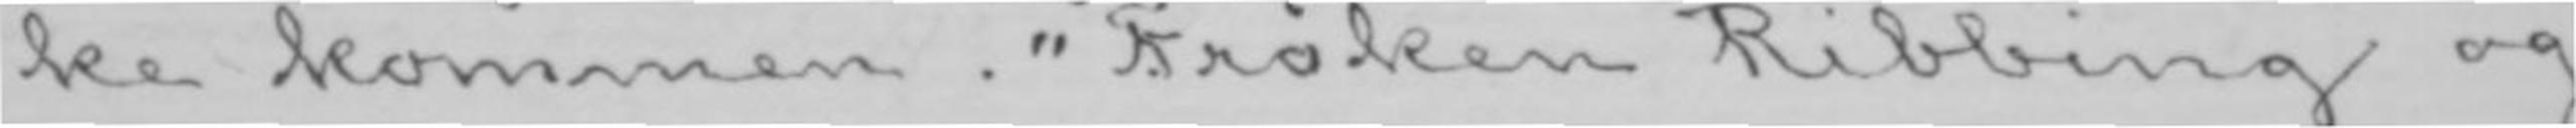ke kommen. «Frøken Ribbing og
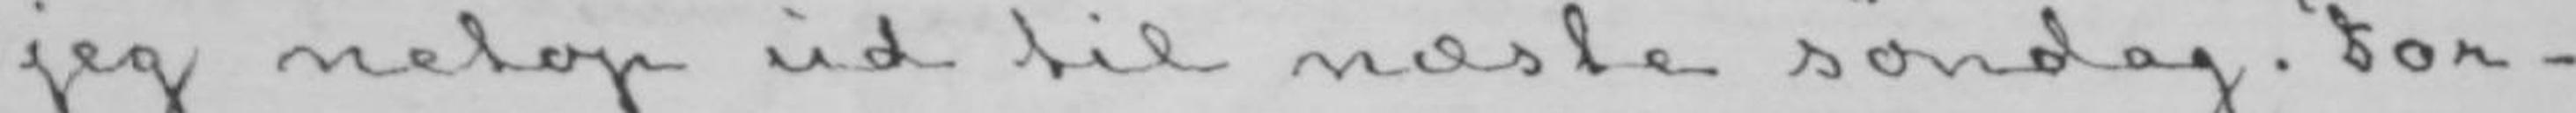jeg netop ud til næste søndag. For-
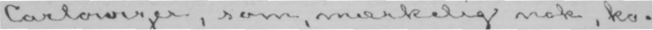Carlowizer, som, mærkelig nok, ko-
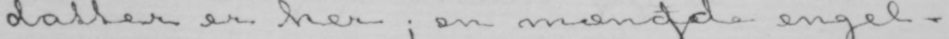datter er her; en mængde engel-
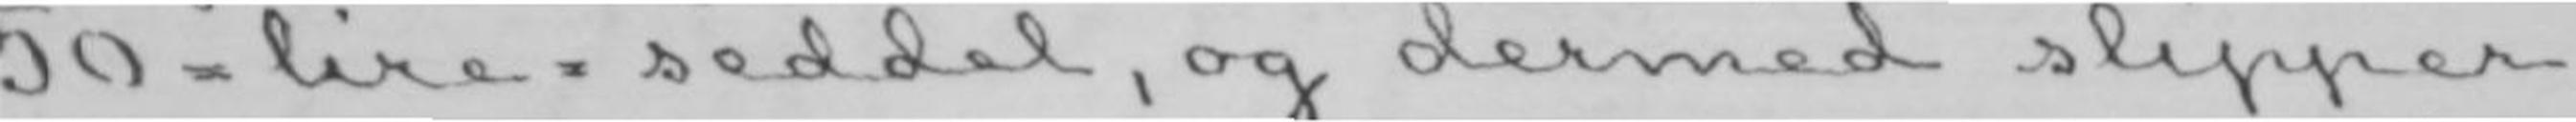50-lire-seddel, og dermed slipper
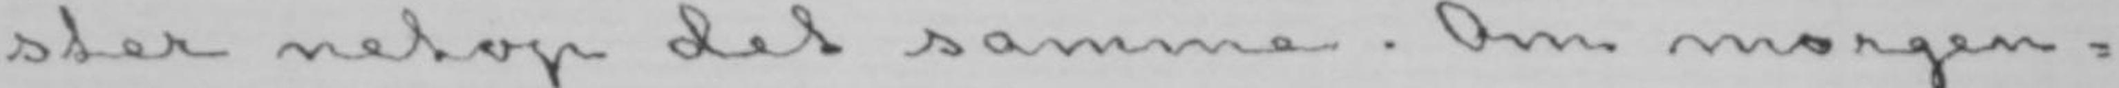ster netop det samme. Om morgen-
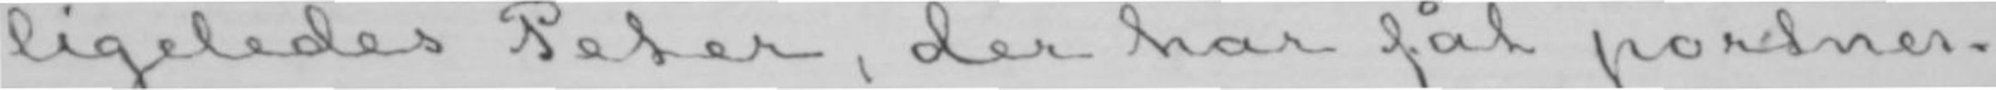ligeledes Peter, der har fåt portner-
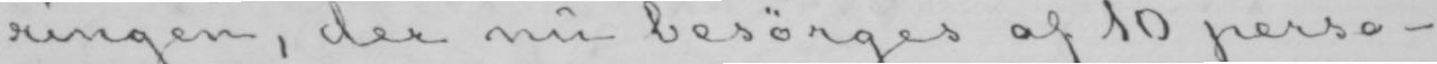ringen, der nu besørges af 10 perso-
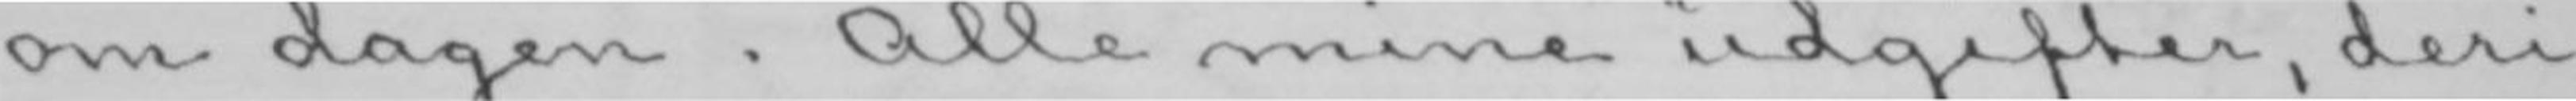om dagen. Alle mine udgifter, deri
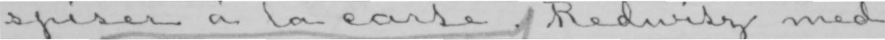spiser a la carte. Redwitz med
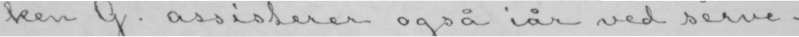ken G. assisterer også iår ved serve-
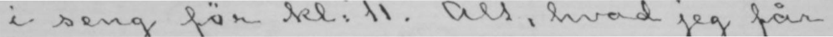i seng før kl: 11. Alt, hvad jeg får
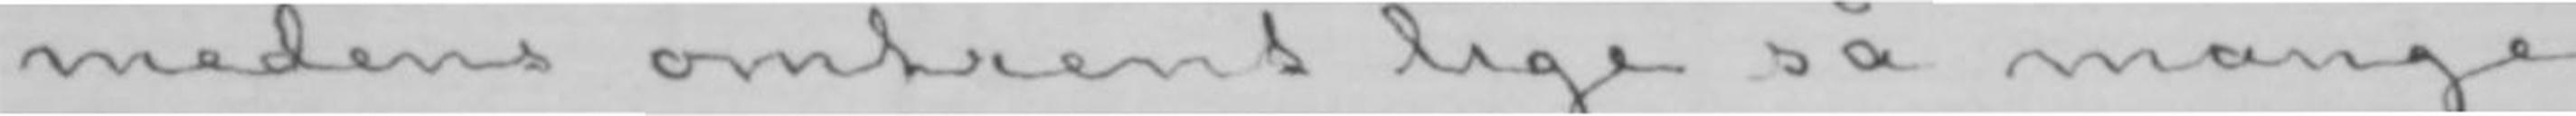medens omtrent lige så mange
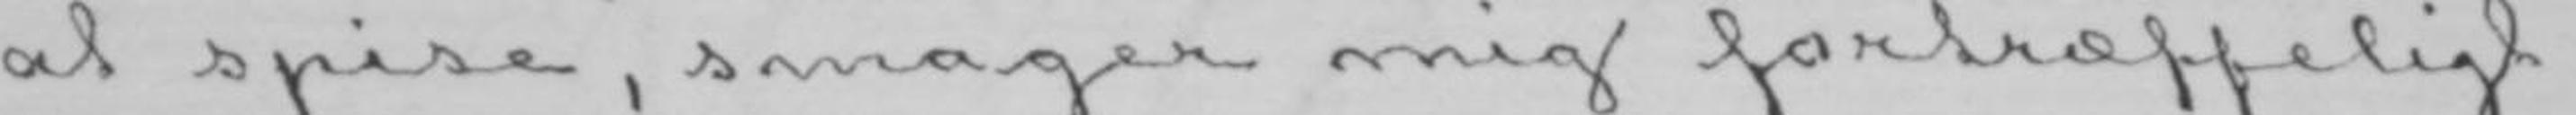at spise, smager mig fortræffeligt.
